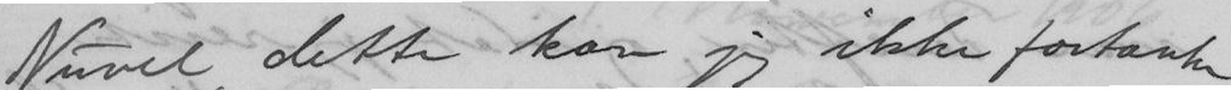Nuvel, dette kan jeg ikke fortænke
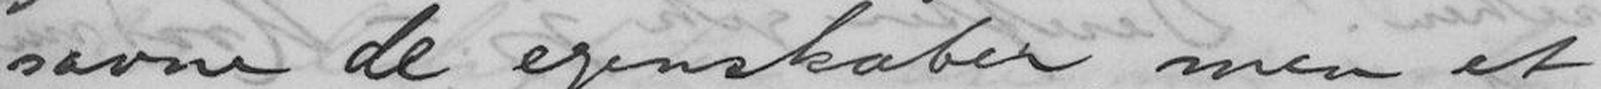savne de egenskaber, men et
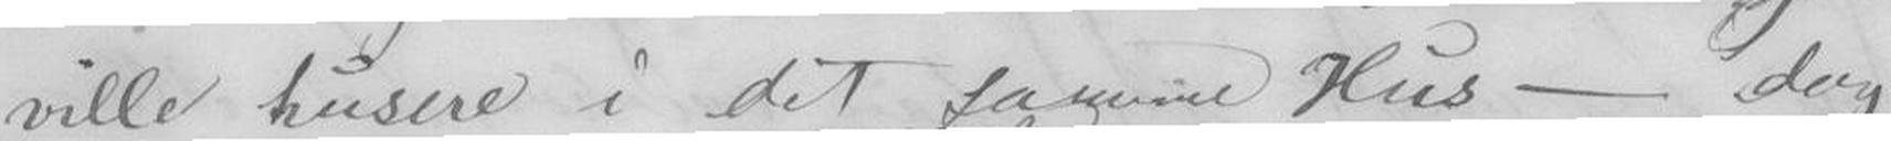ville husere i det samme Hus - dog
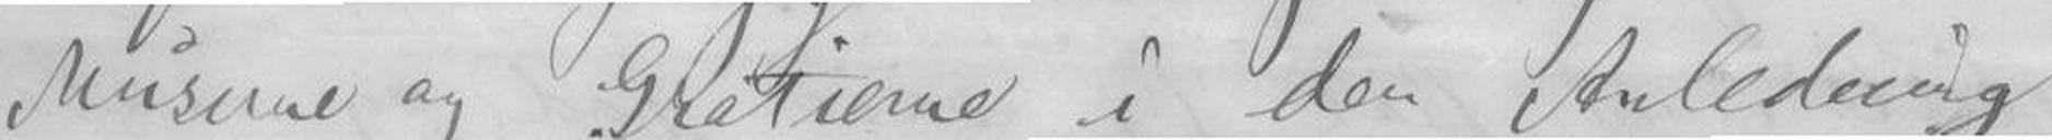Muserne og Gratierne i den Anledning
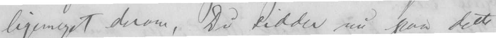ligemeget derom, Du sidder nu paa dette
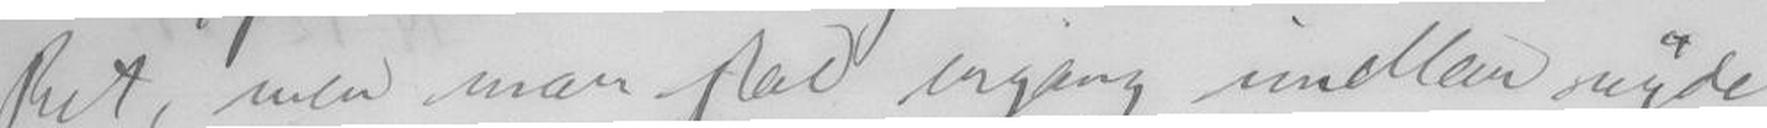Ret, men man skal engang imellem nyde
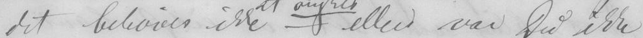det behøves ikke eller var Du ikke
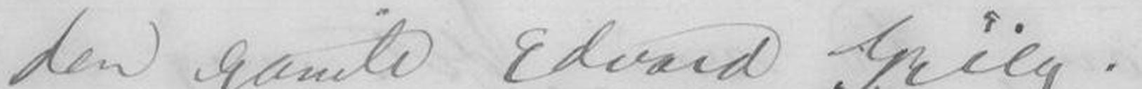den gamle Edvard Grieg.
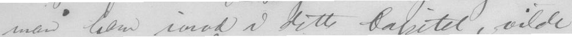man ham imod i dette Capitel, vilde
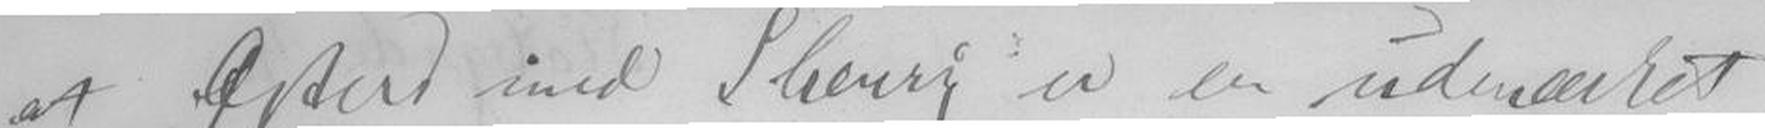at Østers med Sherry er en udmærket
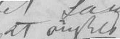at ønskes
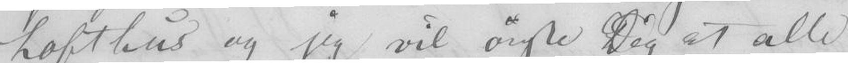Lofthus og jeg vil ønske Dig at alle
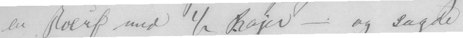en Boeuf med 1/2 Bajer - og sagde
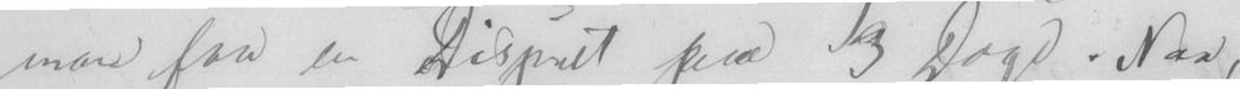man faa en Disput paa 3 Dage. Naa,
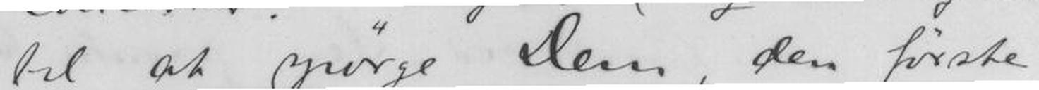til at spørge Dem, den første
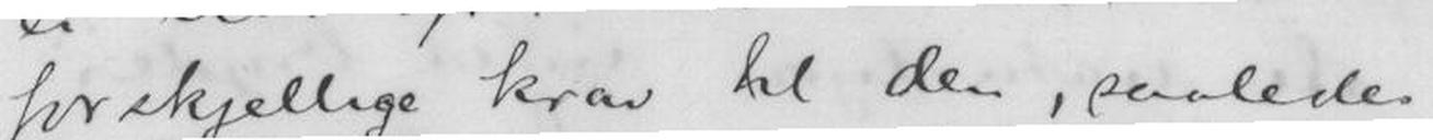forskjellige krav til den, saaledes
